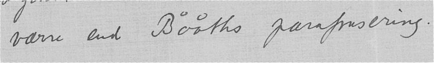værre end Bååths parafrasering.
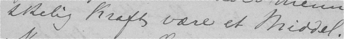skelig kraft være et Middel.
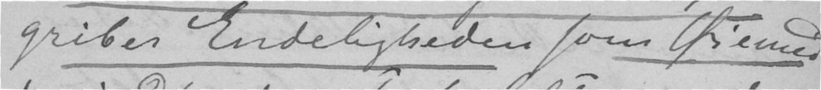griber Endeligheden som Øiemed
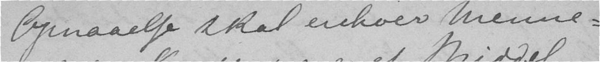Opnaaelse skal enhver menne-
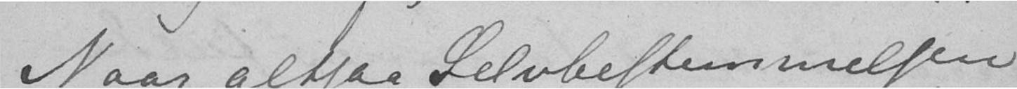Naar altsaa Selvbestemmelsen
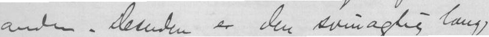anden. Desuden er den svinagtig lang
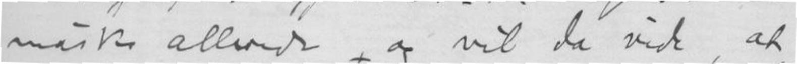måske allerede og vil da vide, at
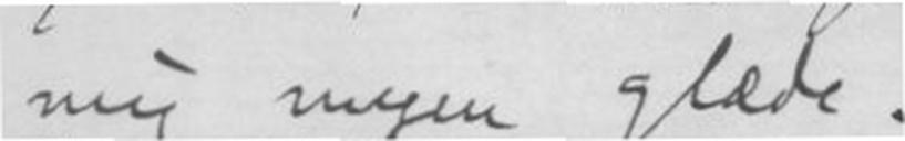mig meget glæde.
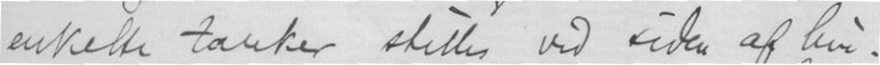enkelte tanker stilles ved siden af hin-
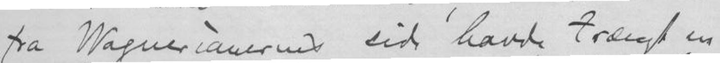fra Wagnerianernes side havde trængt
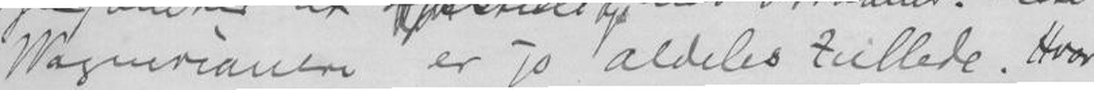Wagnerianere er jo aldeles tullede. Hvor
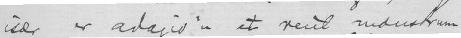især er adagio'n et rent nedustrum
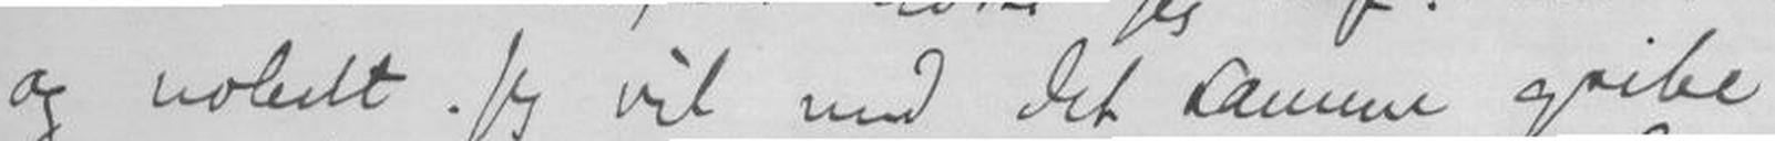og nobelt. Jeg vil med det samme gribe
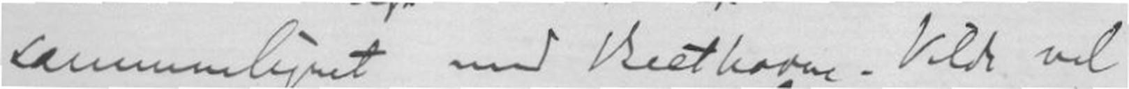sammenlignet med Beethoven. Vilde vel
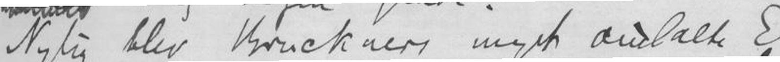Nylig blev Bruckners meget omtalte E-
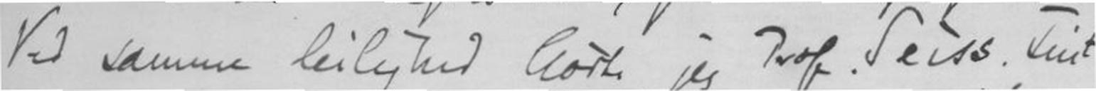Ved samme leilighed hørte jeg Prof. Seiss. Fint
